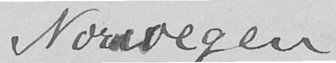Norwegen.
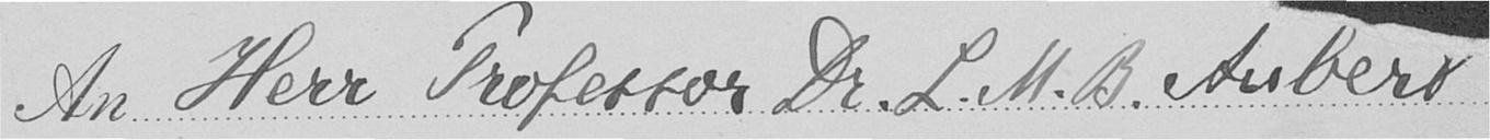An. Herr Professor Dr. L. M. B. Aubert
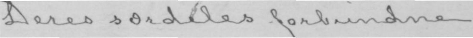Deres særdeles forbundne
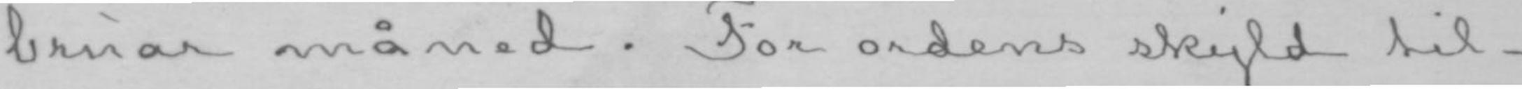bruar måned. For ordens skyld til-
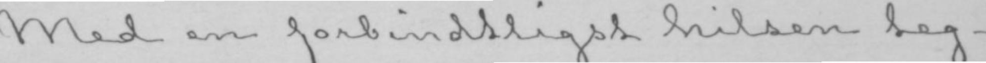Med en forbindtligst hilsen teg-
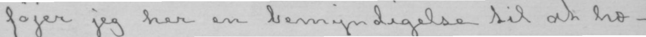føjer jeg her en bemyndigelse til at hæ-
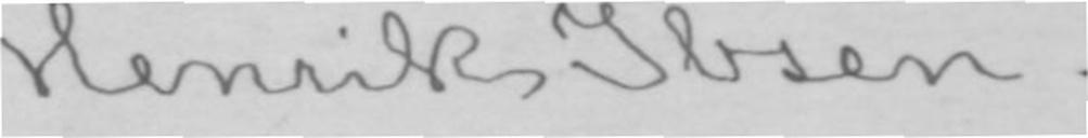Henrik Ibsen.
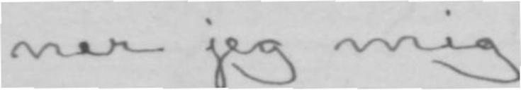ner jeg mig
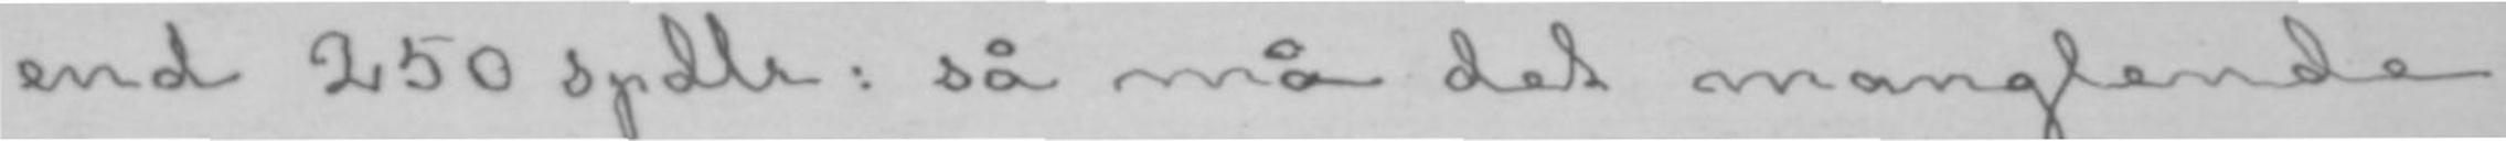end 250 spdlr: så må det manglende
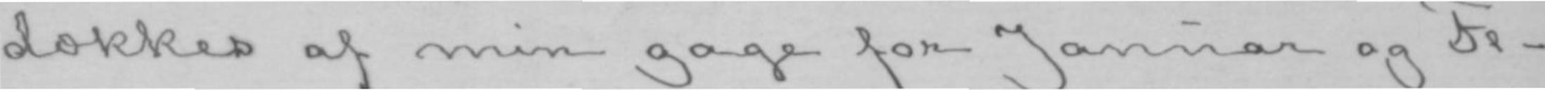dækkes af min gage for Januar og Fe-
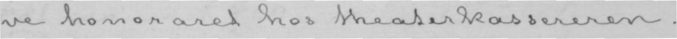ve honoraret hos theaterkassereren.
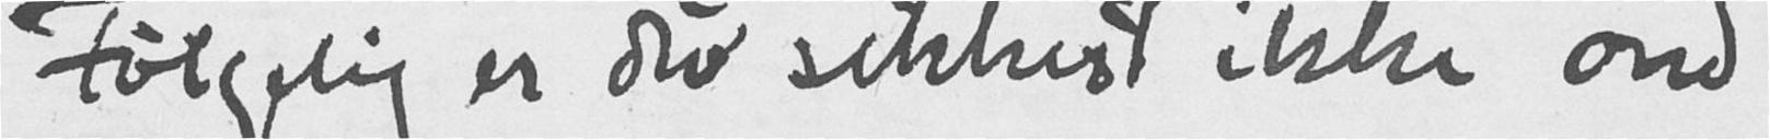Følgelig er du sikkert ikke ond
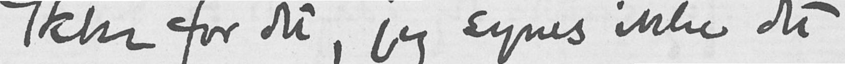Ikke for det, jeg synes ikke det
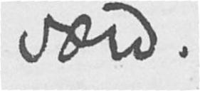værd.
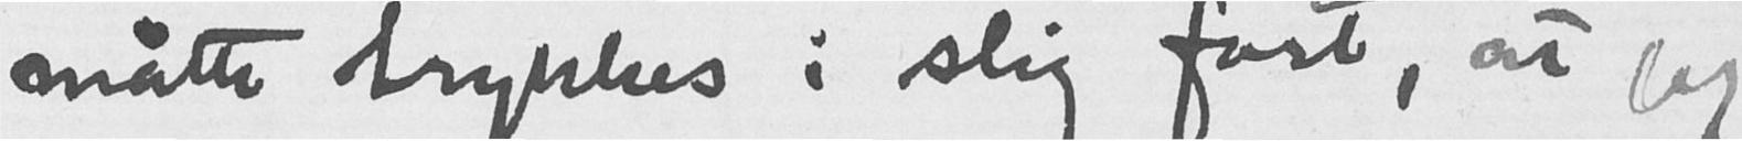måtte trykkes i slig fart, at jeg
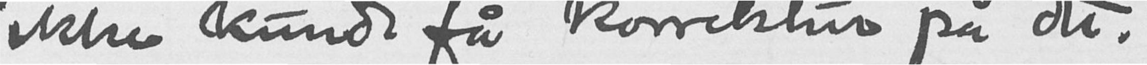ikke kunde få korrektur på det.
